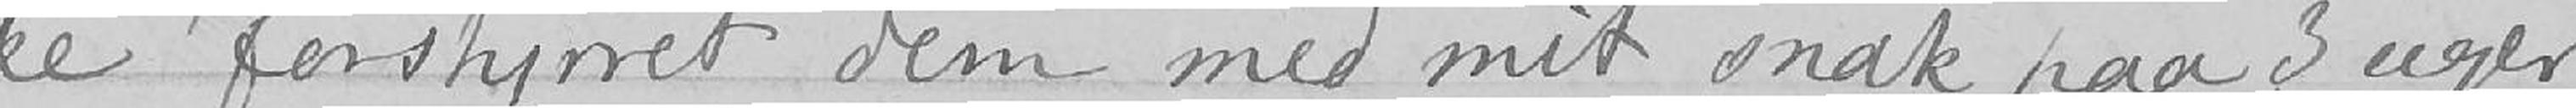ke forstyrret dem med mit snak paa 3 uger.
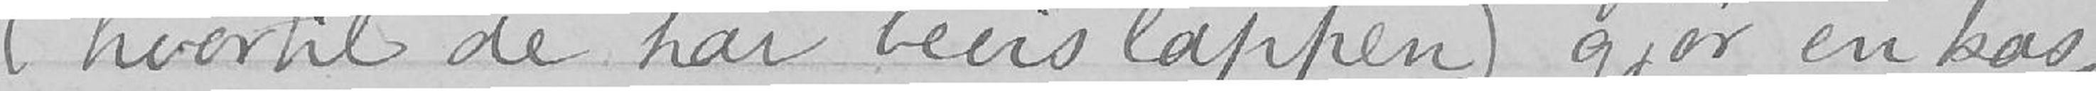(hvortil de har bevislappen) gjør en kas-
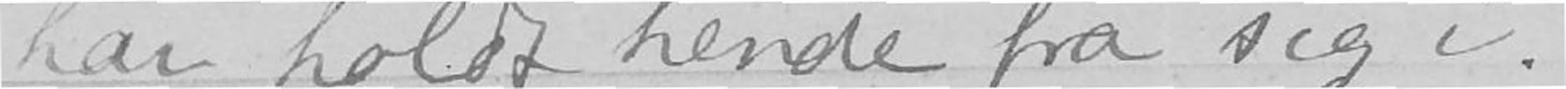har holdt hende fra sig i.
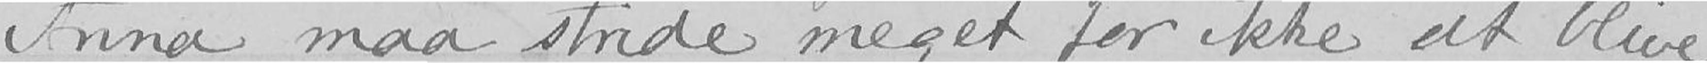Anna maa stride meget for ikke at blive
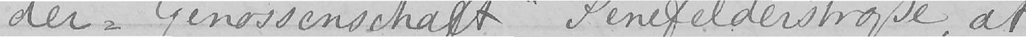Genossenschaft» Senefelderstraße, at
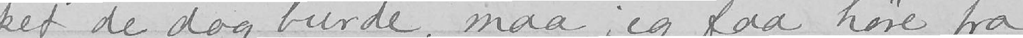ket de dog burde, maa jeg faa høre fra
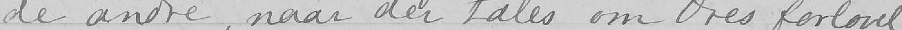de andre, naar der tales om Oves forlovel
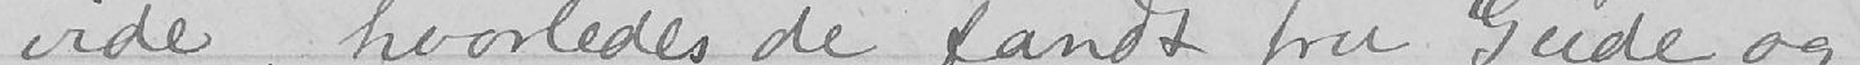vide, hvorledes de fandt fru Gude og
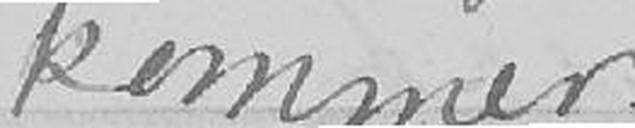kommer.
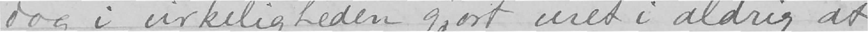dog i virkeligheden gjort uret i aldrig at
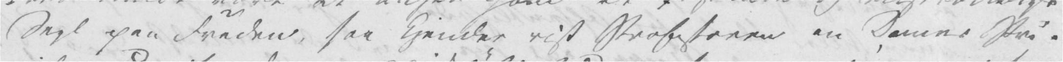Segl paa Freden, saa kjender vist Professoren en Dames Pri-
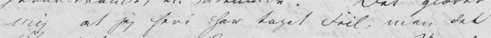mig at jeg heri har taget Feil, men det
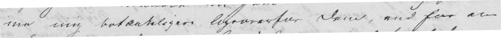me mig betænkeligere ligeoverfor Dem, end for en
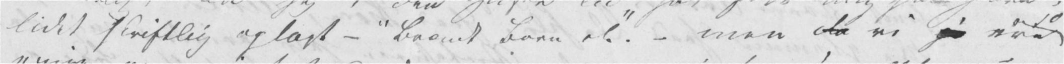lidet skriftlig oplagt – «Brændt Barn etc». – men da vi jo ere
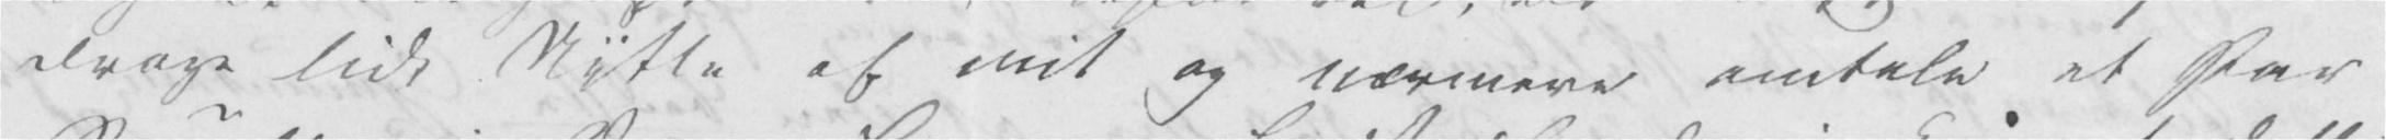drage lidt Nytte af mit og nærmere omtale et Par
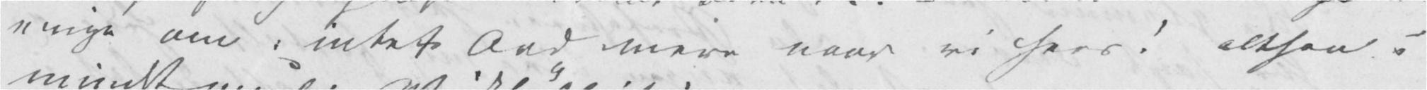jo enige om: intet Ord mere naar vi sees! altsaa:
